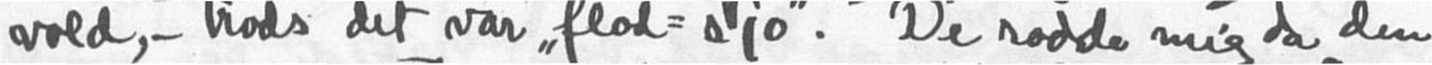vold, - trods det var "flod-sjø". De rodde mig da den
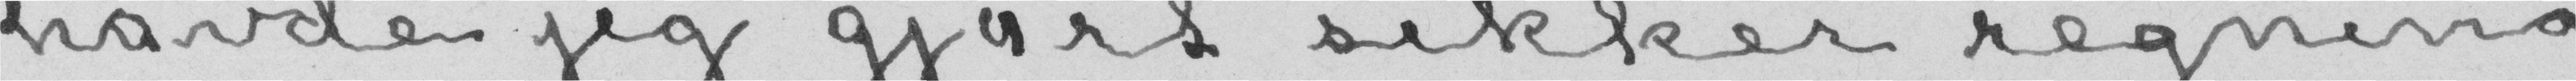havde jeg gjort sikker regning
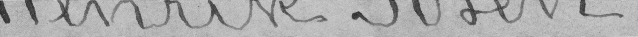Henrik Ibsen.
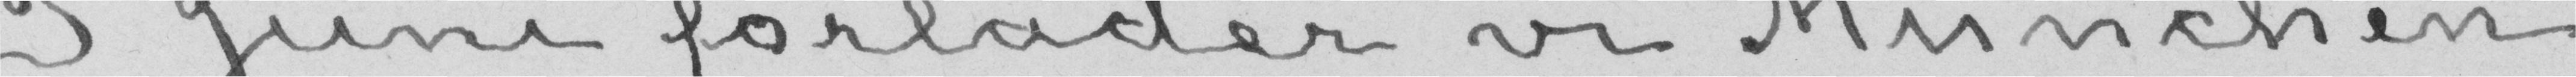I Juni forlader vi München
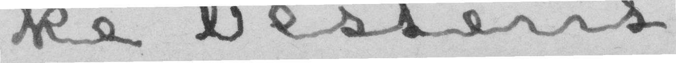ke bestent.
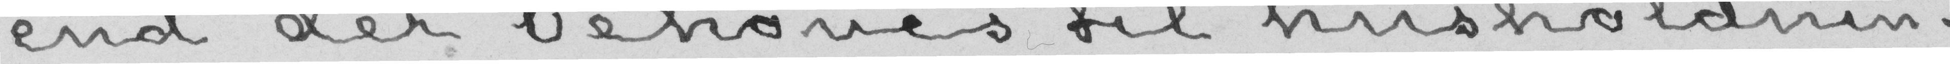end der behøves til husholdnin-
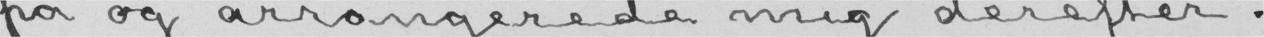på og arrangerede mig derefter.
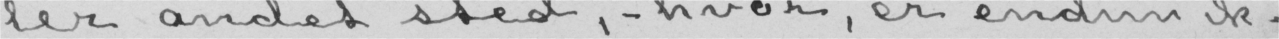ler andet sted,- hvor, er endnu ik-
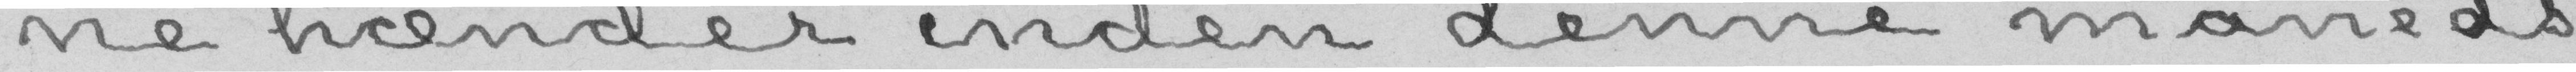ne hænder inden denne måneds
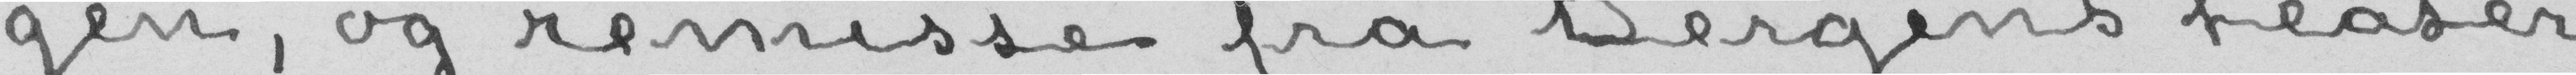gen, og remisse fra Bergens teater
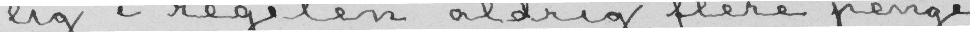lig i regelen aldrig flere penge
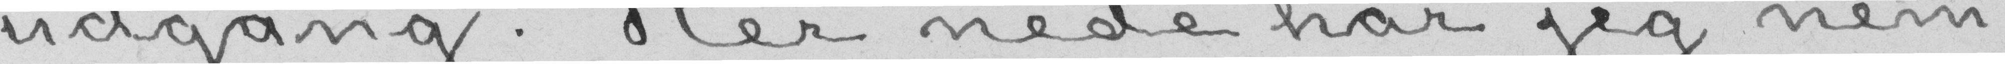udgang. Her nede har jeg nem-
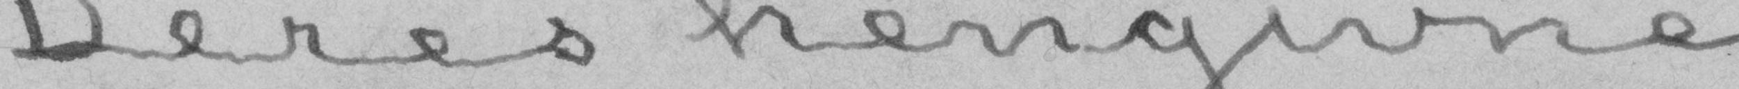Deres hengivne
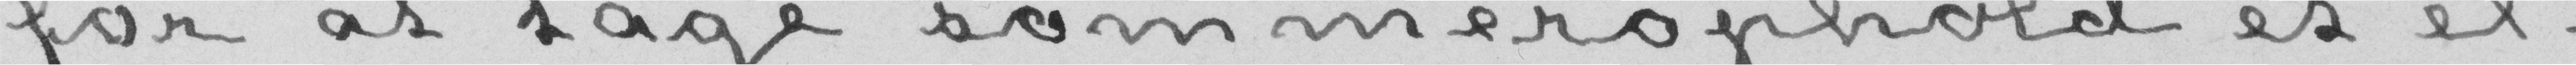for at tage sommerophold et el-
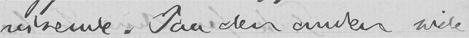visende. Paa den anden side
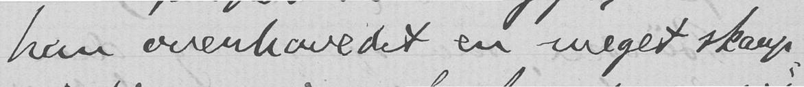han overhovedet en meget skarp-
